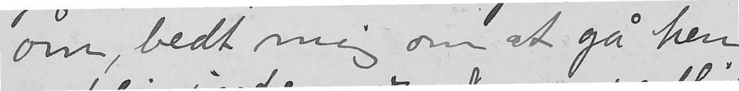om, bedt mig om at gå hen
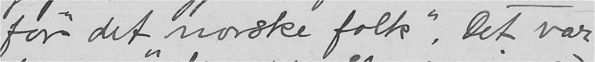for det "norske folk". Det var
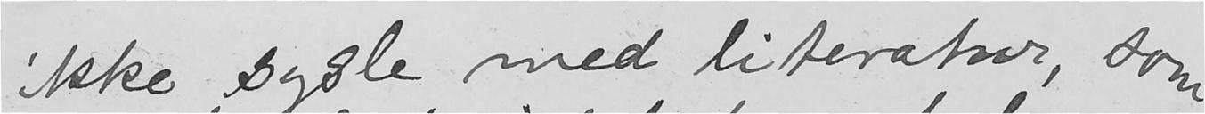ikke sysle med literatur, som
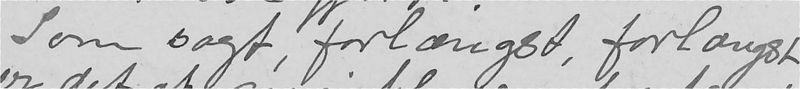Som sagt, forlængst, forlængst
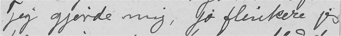jeg gjorde mig, lo flinkere jeg
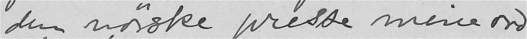den norske presse mine ord
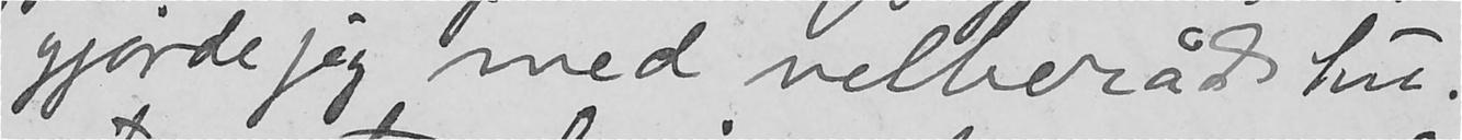gjorde jeg med velberåd hu.
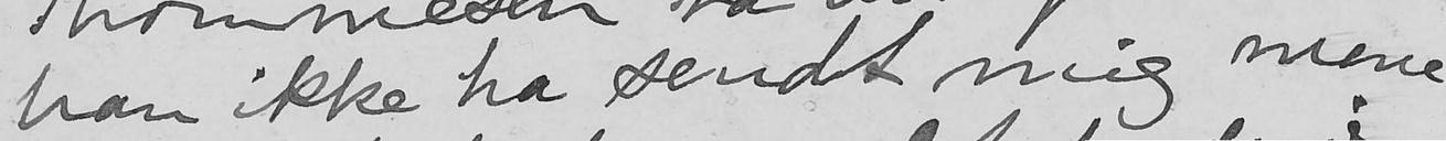han ikke ha sendt mig mine
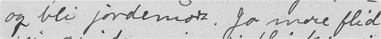og bli jordemod. Jo mere flid
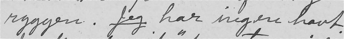ryggen. Jeg har ingen havt.
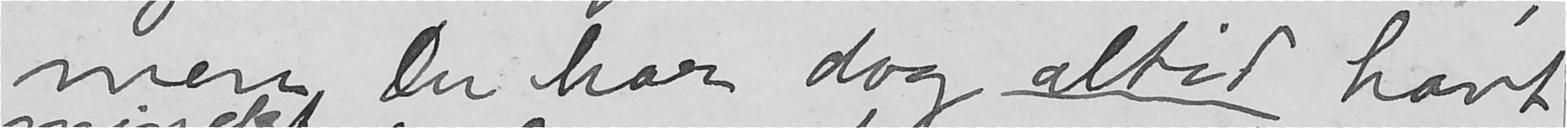men Du har dog altid havt
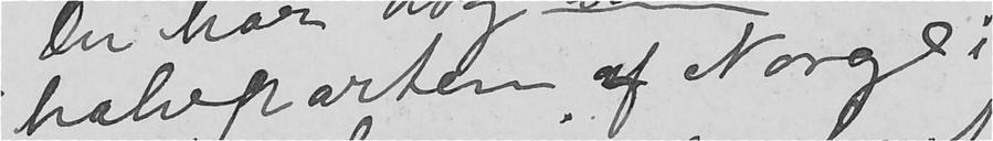halvparten af Norge i
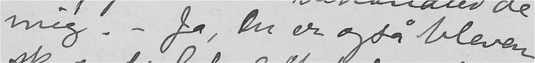mig. - Ja, Du er også bleven
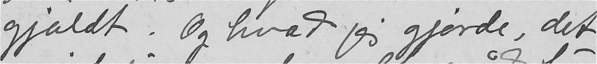gjaldt. Og hvad jeg gjorde, det
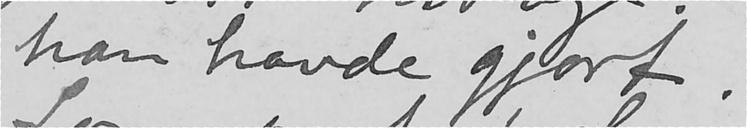han havde gjort.
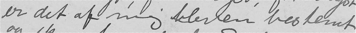er det af mig bleven bestemt
